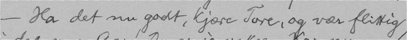- Ha det nu godt, kjære Tore, og vær flittig
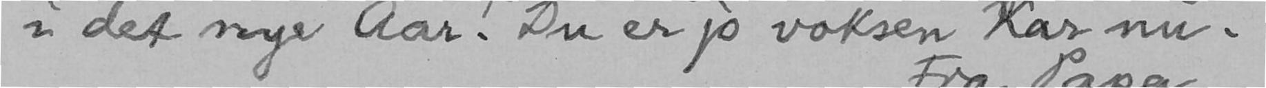i det nye Aar! Du er jo voksen Kar nu.
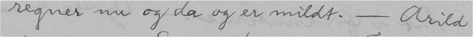regner nu og da og er mildt. - Arild
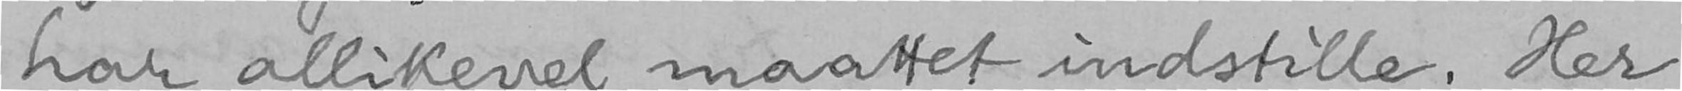har allikevel maattet indstille. Her
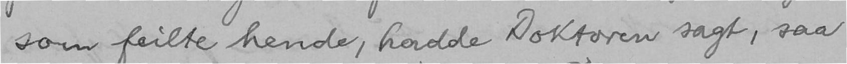som feilte hende, hadde Doktoren sagt, saa
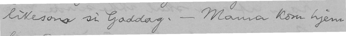likesom si Goddag. - Mama kom hjem
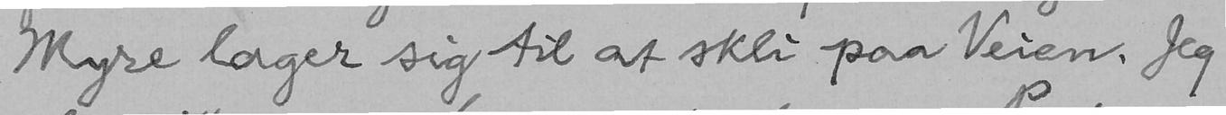Myre lager sig til at skli paa Veien. Jeg
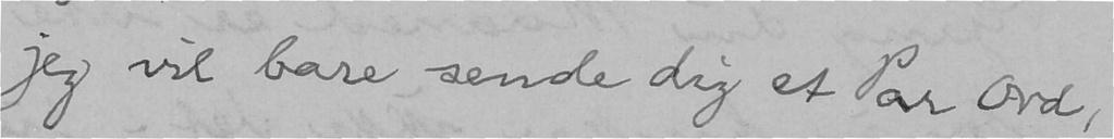jeg vil bare sende dig et Par Ord,
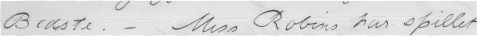Bedste. - Miss Robins har spillet
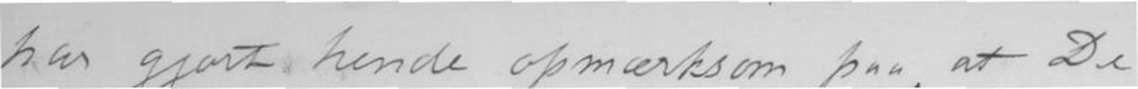har gjort hende opmærksom paa, at De
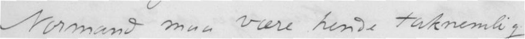Normand maa være hende taknemlig
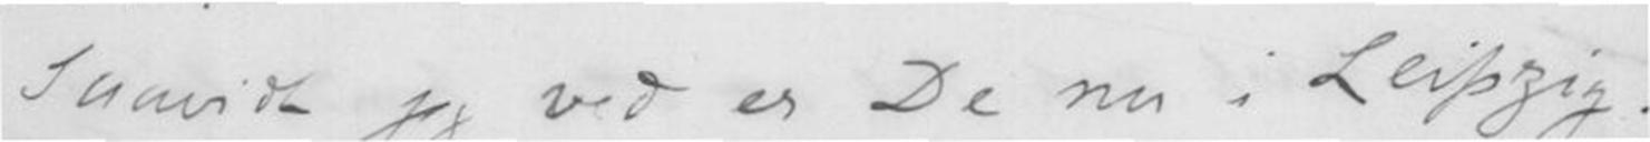Saavidt jeg ved er De nu i Leipzig.
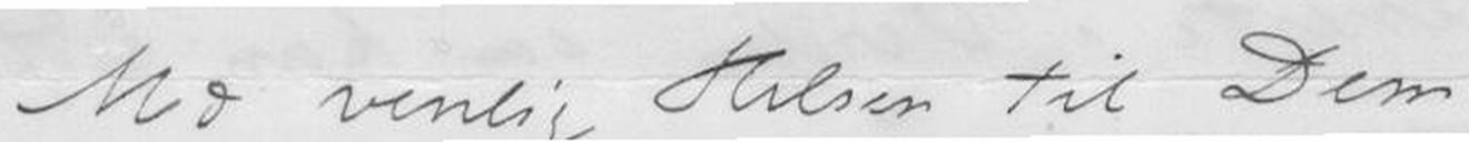Med venlig Hilsen til Dem
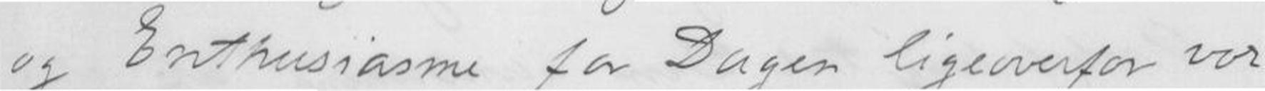og Enthusiasme for Dagen ligeoverfor vor
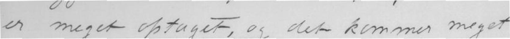er meget optaget, og det kommer meget
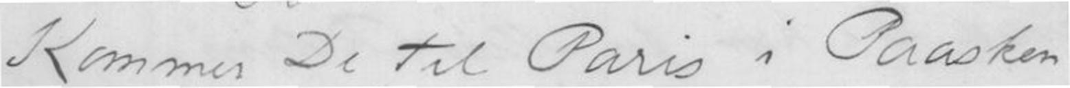Kommer De til Paris i Paasken
